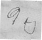dog
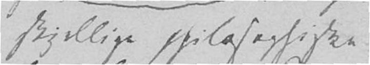skjellige philosophiske
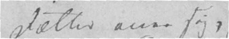Fælles over sig,
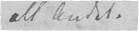alt Andet.
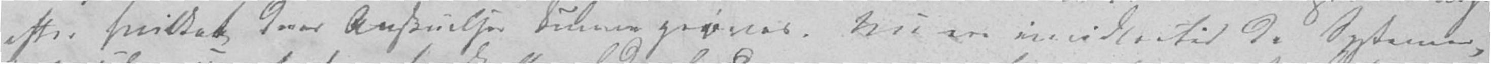efter hvilket deres Anskuelse kunne prøves. Nu ere imidlertid de Systemer,
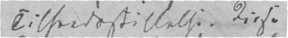Tilfredsstillelse. Disse
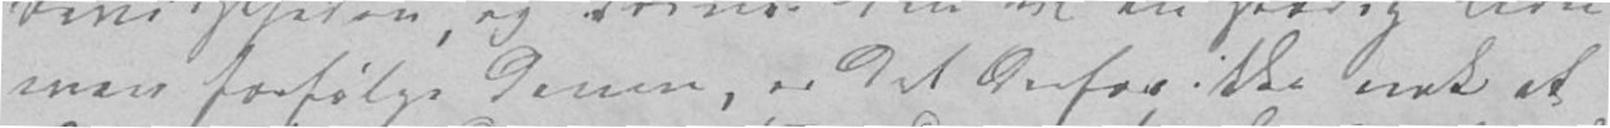man forfølge denne, er det derfor ikke nok at
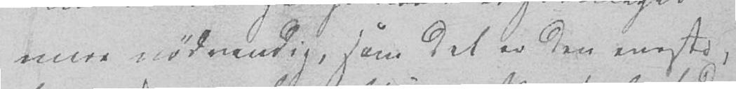mere nødvendig, som det er den eneste,
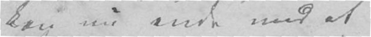kan nu ende med at
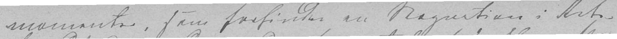momenter, som forefinder en Stagnation i Rets-
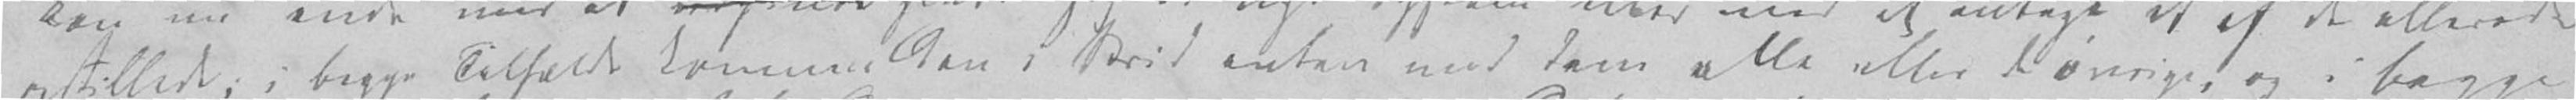opstillede, i begge Tilfælde kommer den i Strid enten med dem alle eller de øvrige, og i begge
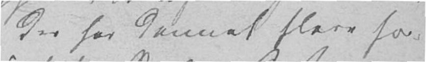der har dannet flere for-
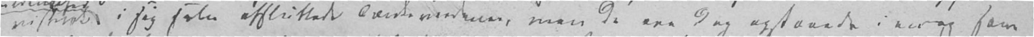vistnok i sig selv afsluttede Tænkeverdener, men de ere dog opstaaede i X sam-
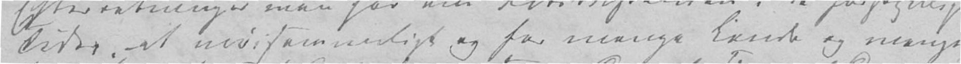Tider, et møisommeligt og for mange Lande og mange
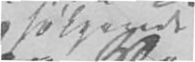følgende
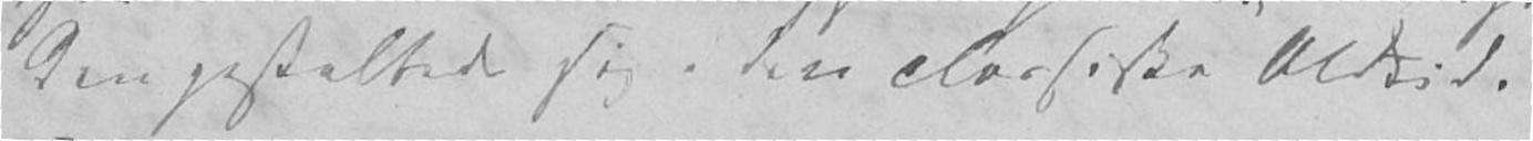den gestaltede sig i den classiske Oldtid.
